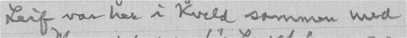Leif var her i Kveld sammen med
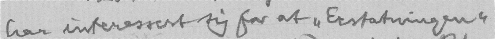har interessert sig for at "Erstatningen"
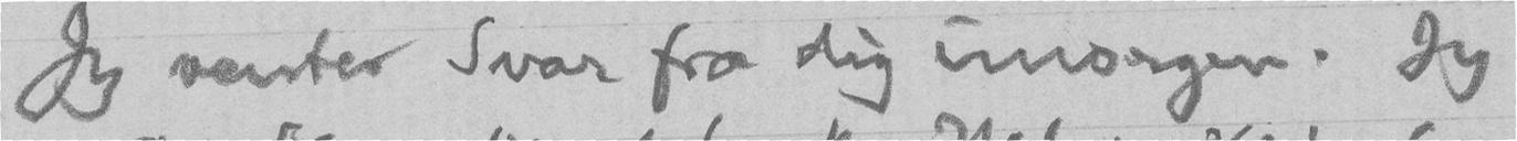Jeg venter Svar fra dig imorgen. Jeg
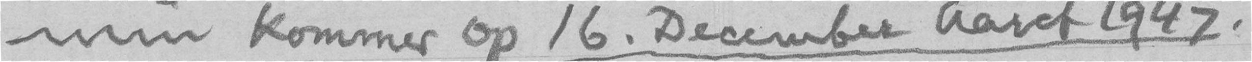min kommer op 16. December Aaret 1947.
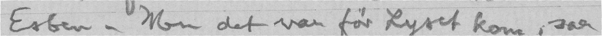Esben. Men det var før Lyset kom, saa
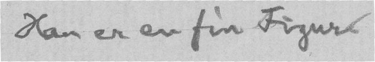Han er en fin Figur.
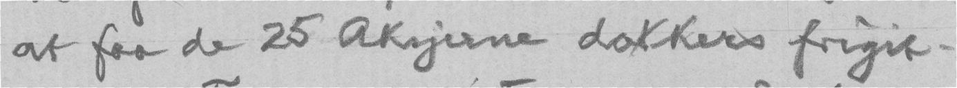at faa de 25 Aksjerne dokkers frigit.
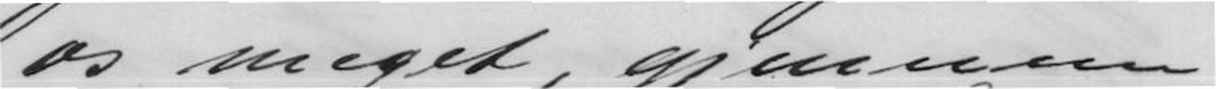os meget, gjennem
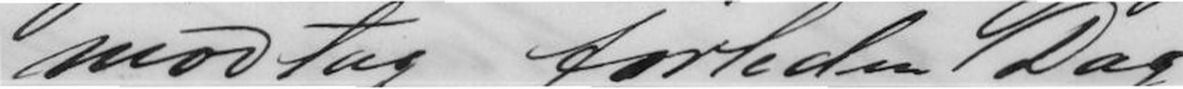modtog forleden Dag;
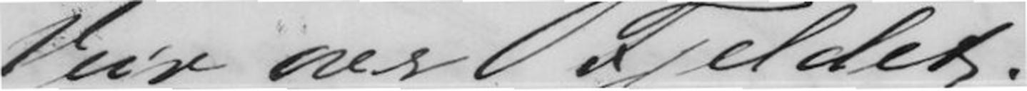Veir over Fjeldet.
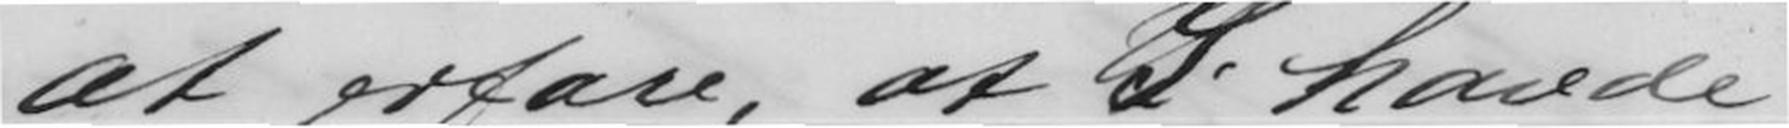at erfare, at I havde
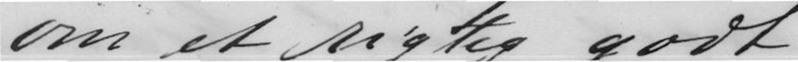om et rigtig godt
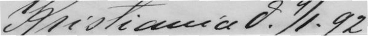Kristiania d. 9/1. 92
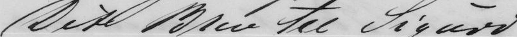Dit Brev til Sigurd
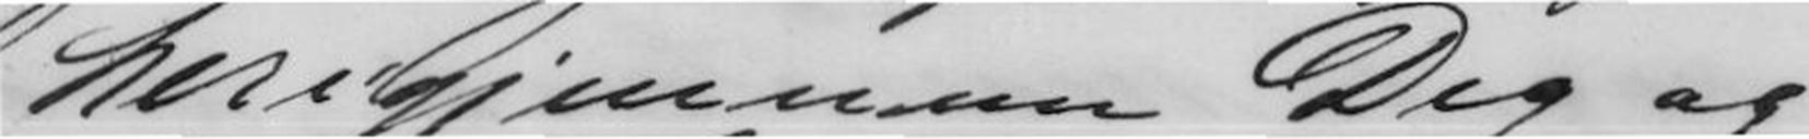herigjennem Dig og
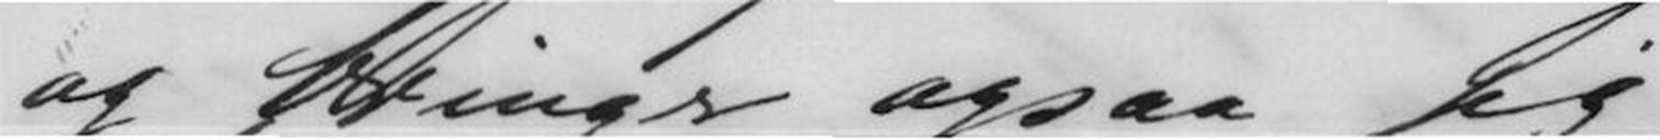og bringer ogsaa jeg
